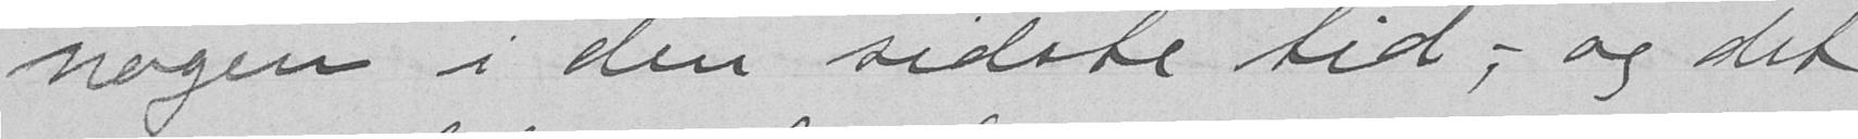nogen i den sidste tid, - og det
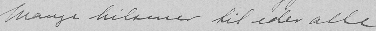Mange hilsener til eder alle
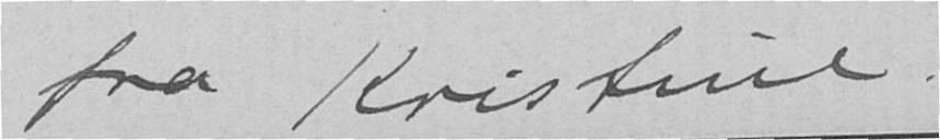fra Kristine.
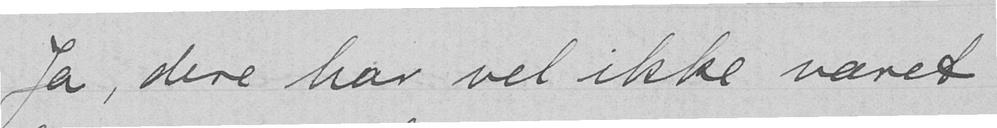Ja, dere har vel ikke været
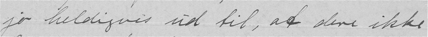jo heldigvis ud til, at dere ikke
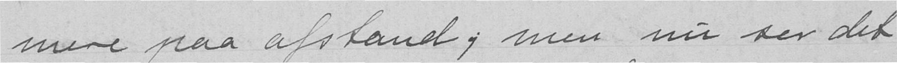mere paa afstand; men nu ser det
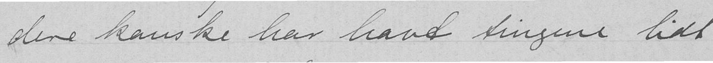dere kanske har havt tingene lidt
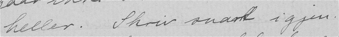heller. Skriv snart igjen!
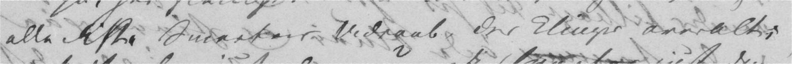alle disse Smertens Udraab, der klinger overalt;
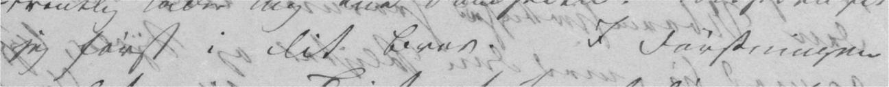jeg først i Dit Brev. I Førstningen
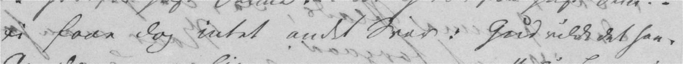vi faae dog intet andet Svar: Gud vilde det saa.
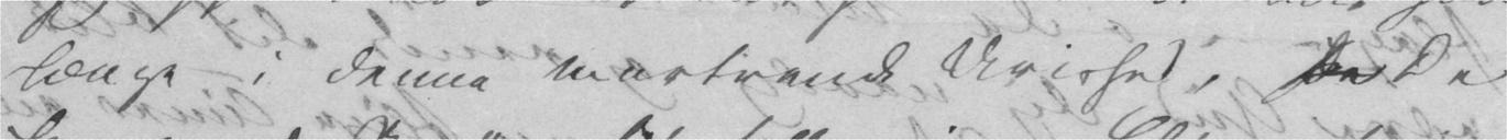længe i denne martrende Uvished, De
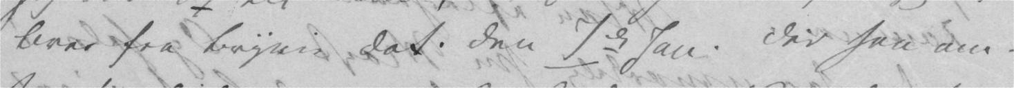Brev fra Brynie dat. den 7de Jan. der han om-
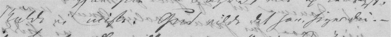skulde vi miste. Gud vilde det saa, siger Du. –
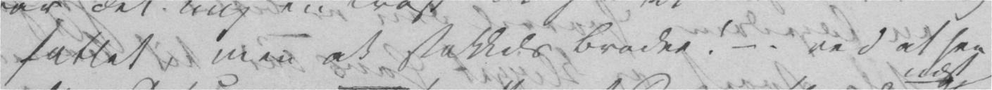fattet, men ak stakkels Broder! – – ved at see
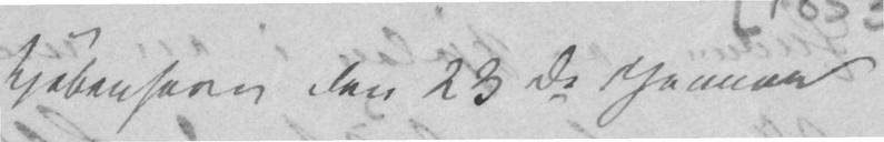Kjøbenhavn den 23de Januar
